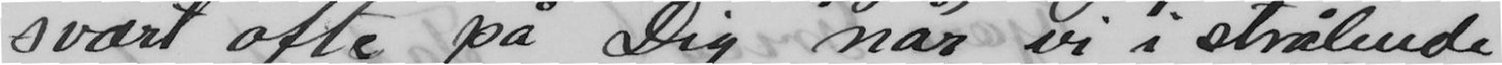svært ofte på Dig når vi i strålende
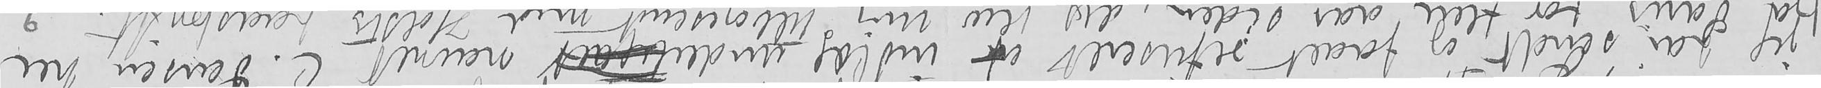jeg har sendt og faaet refuseret et indlæg undertegnet navnet C. Jensen her
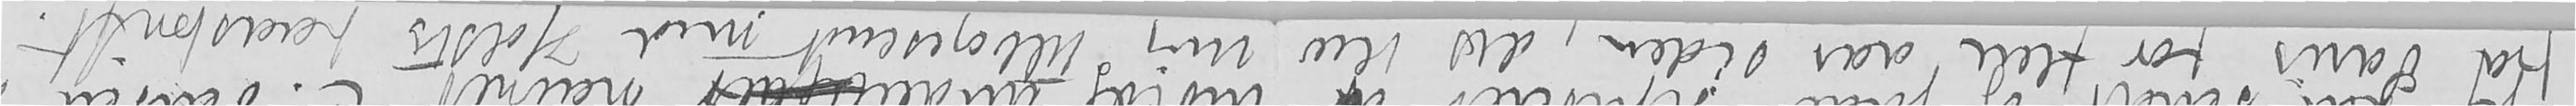fra Paris for flere aar siden, det blev mig tilbagesendt med Holsts paaskrift.
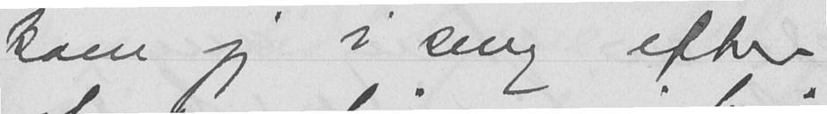kom jeg i seng efter
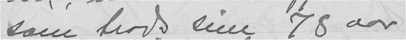som trods sine 78 aar
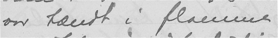var tændt i flamme
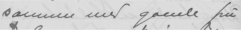sammen med gamle fru
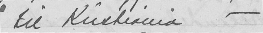til Kristiania -
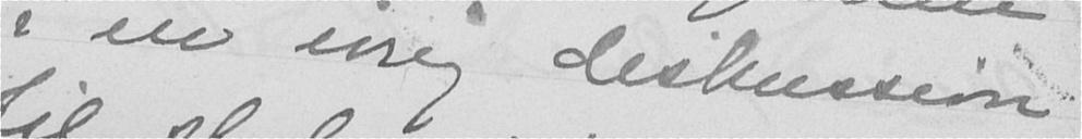i en ivrig diskussion
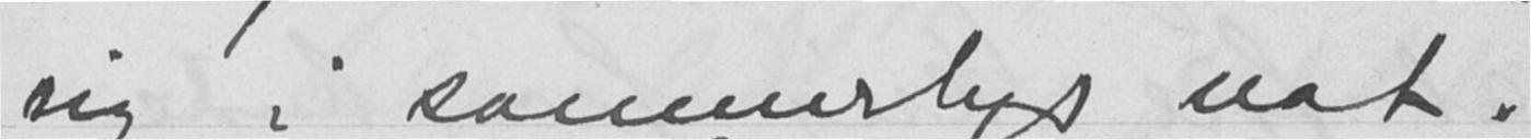rig i sommerlys nat.
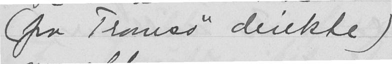(fra Tromsø direkte)
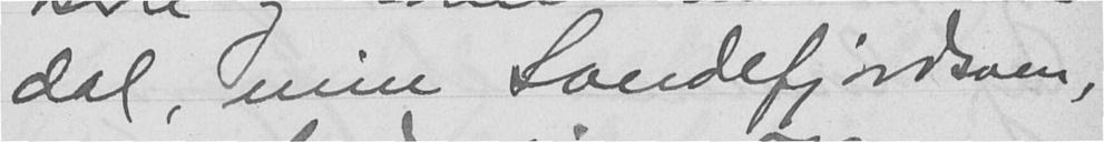dal, min Sandefjordsven,
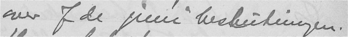over 7de juni beslutningen.
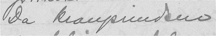Da kronprindsen
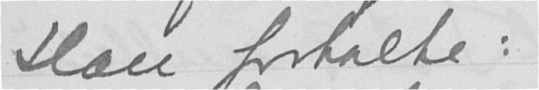Han fortalte:
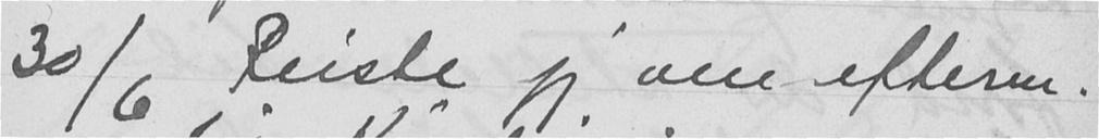30/6 Reiste jeg om efterm.
